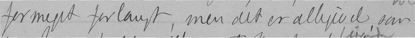formeget forlangt, men det er alligevel som
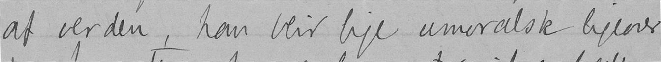af verden, han blir lige umoralsk ligeover
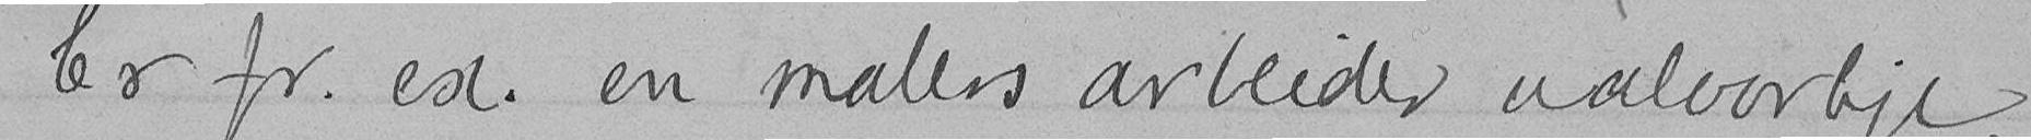Er fr. ex. en malers arbeider ualvorlige,
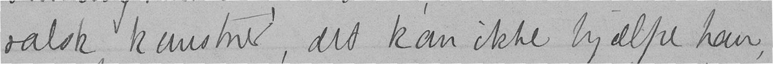ralsk kunstner, det kan ikke hjælpe ham,
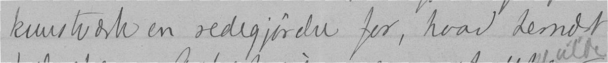kunstværk en redegjørelse for, hvad dernæst
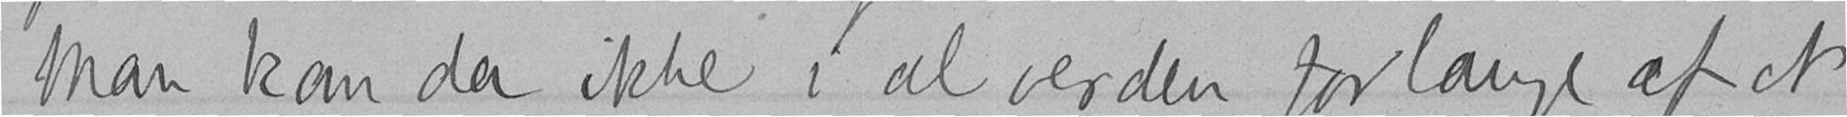Man kan da ikke i al verden forlange af et
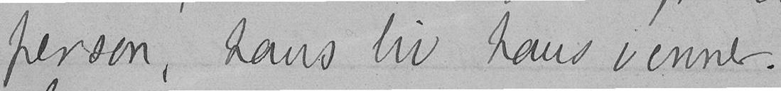person, hans liv hans venner.
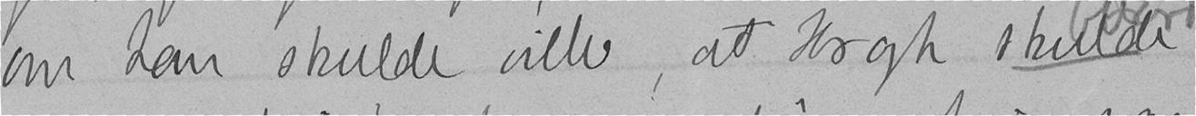om han skulde ville, at Krogh skulde
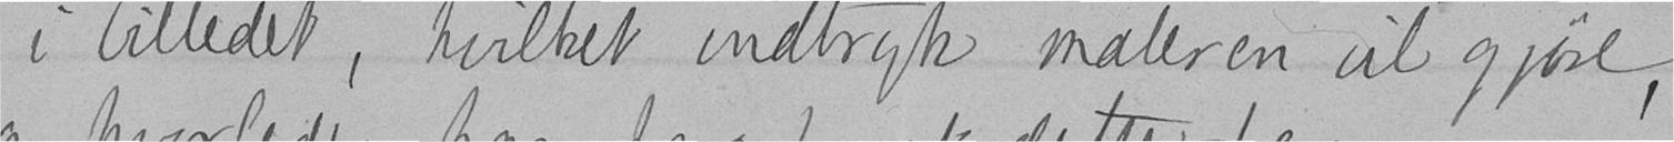i billedet, hvilket indtryk maleren vil gjøre,
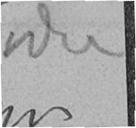være
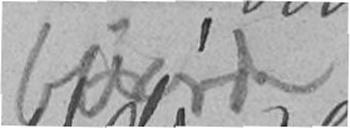burde
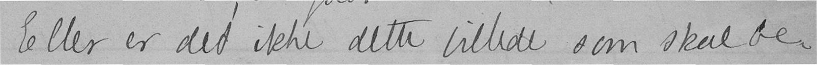Eller er det ikke dette billede som skal be-
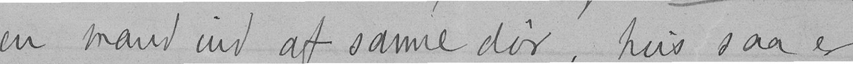en mand ind af samme dør, hvis saa er
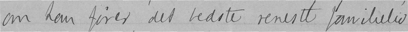om han fører det bedste reneste familieliv
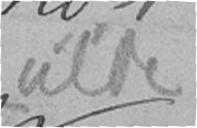vilde
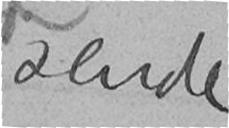sende
